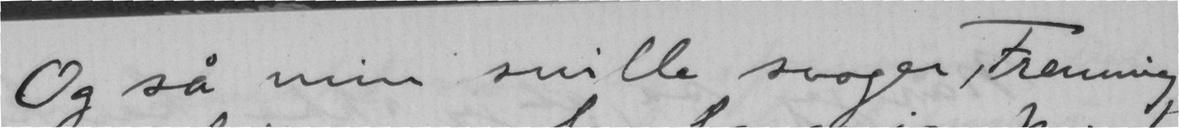Og så min snille svoger, Frenning,
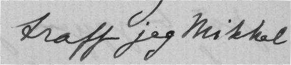traff jeg Mikkel
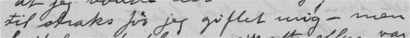til straks før jeg giflet mig - men
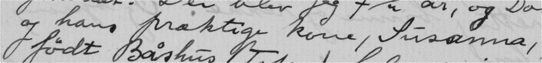og hans præktige kone, Susanna,
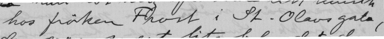hos frøken Frost i St. Olavs gate,
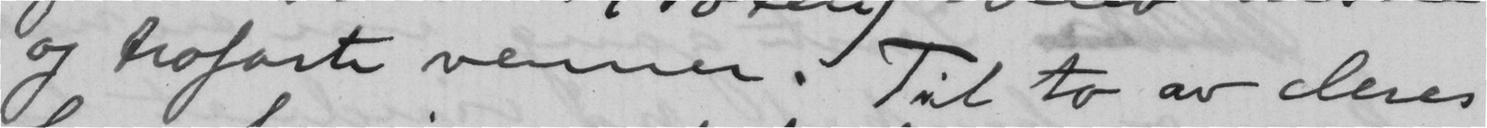og trofaste venner. Til to av deres
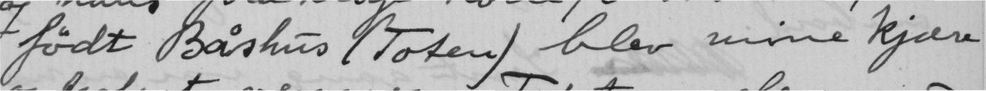født Båshus (Toten) blev mine kjære,
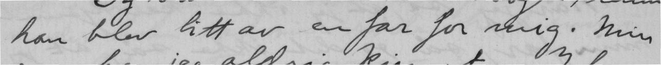han blev litt av en far for mig. Min
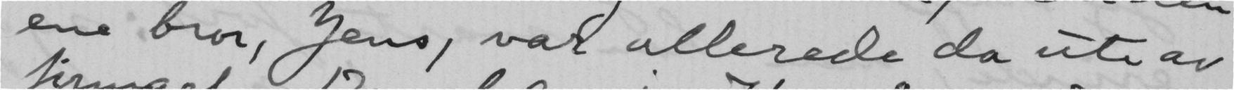ene bror, Jens, var allerede da ute av
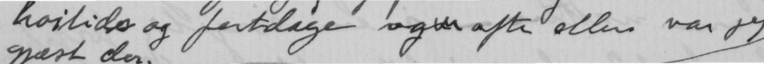høitids og fæstdage, og  ofte ellers var jeg
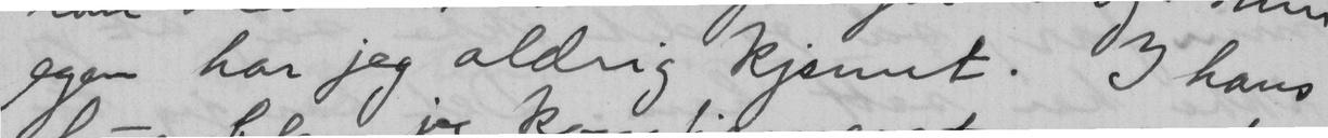egen har jeg aldrig kjennt. I hans
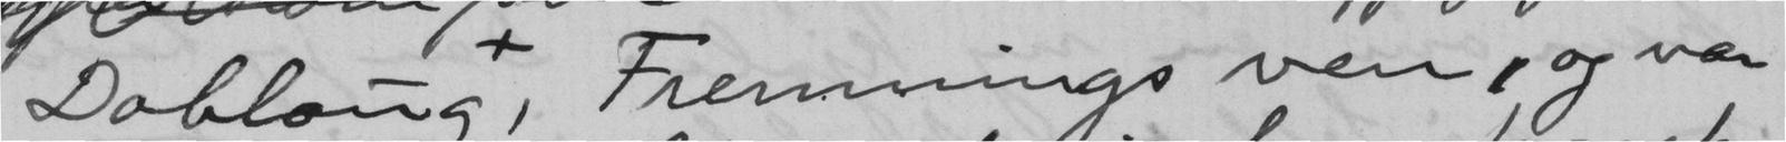Doblaug, Frennings ven, og var
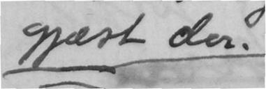gjæst der.
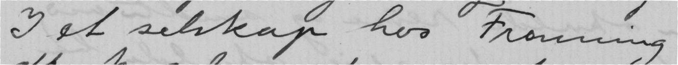I et selskap hos Frenning
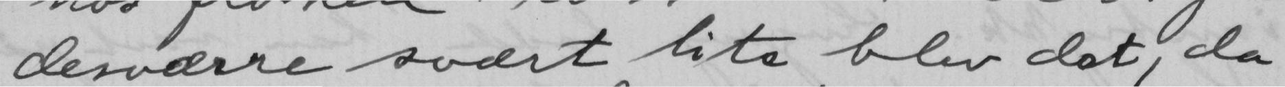desværre svært lite blev det, da
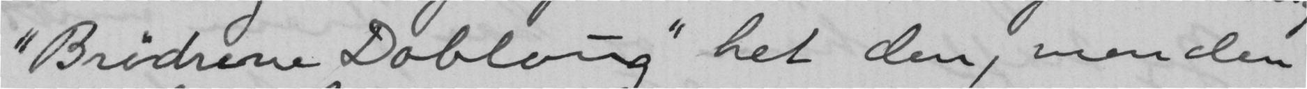"Brødrene Dobloug" het den, men den
